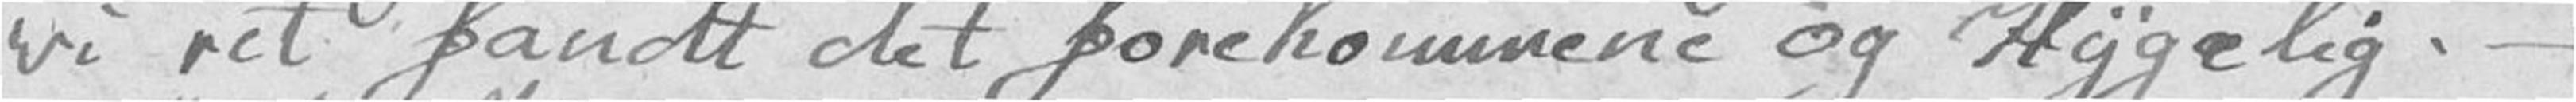vi ret fandt det forekommene og Hygelig. –
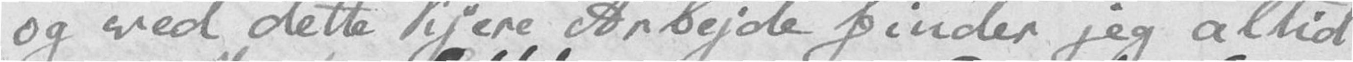og ved dette kjere Arbejde finder jeg altid
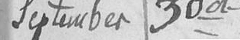September 30d.
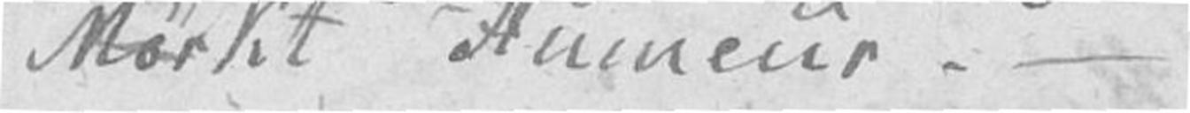Mørkt Humeur. –
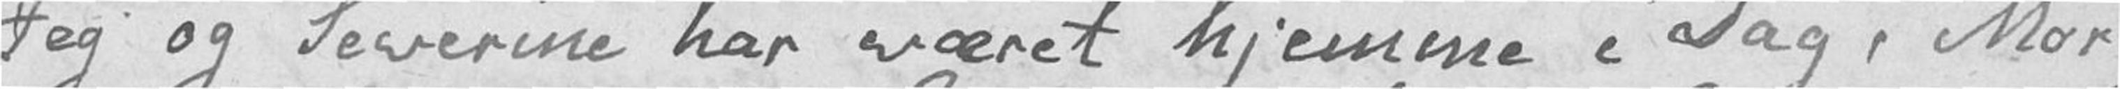Jeg og Severine har været hjemme i Dag, Mor
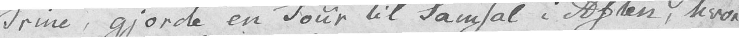Trine, gjorde en Tour til Samsal i Aften, hvor
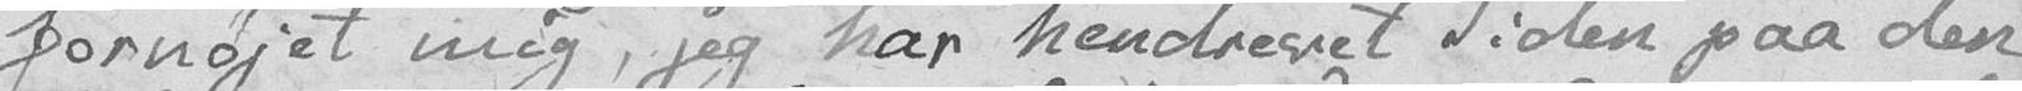fornøjet mig, jeg har hendrevet Tiden paa den
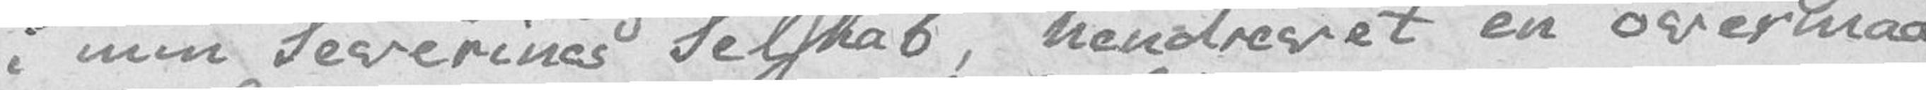i min Severines Selskab hendrevet en overmaa
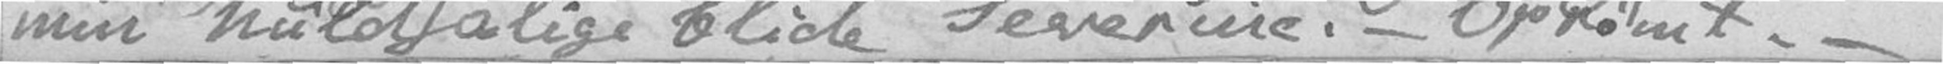min huldsalige blide Severine. – Oprømt. –
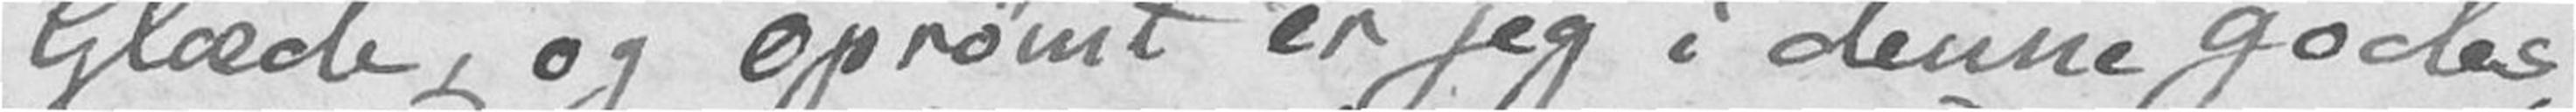Glæde, og oprømt er jeg i denne godes
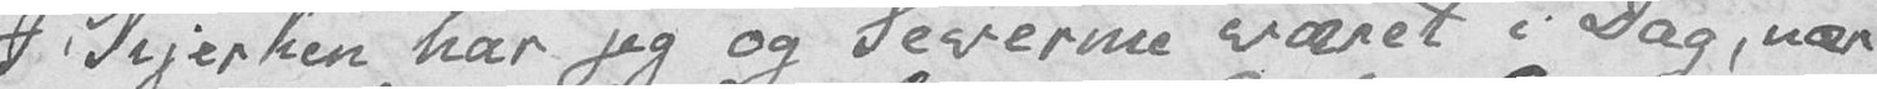I Kjerken har jeg og Severine været i Dag, nær
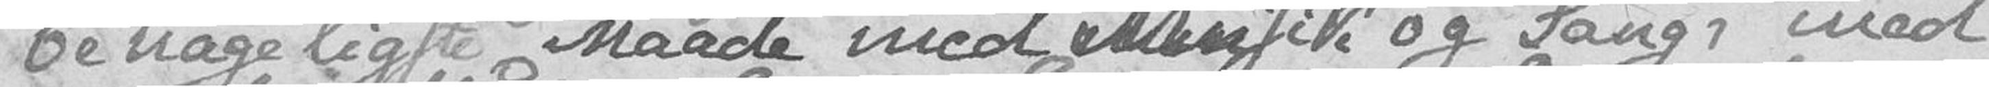behageligste Maade med Musik og Sang, med
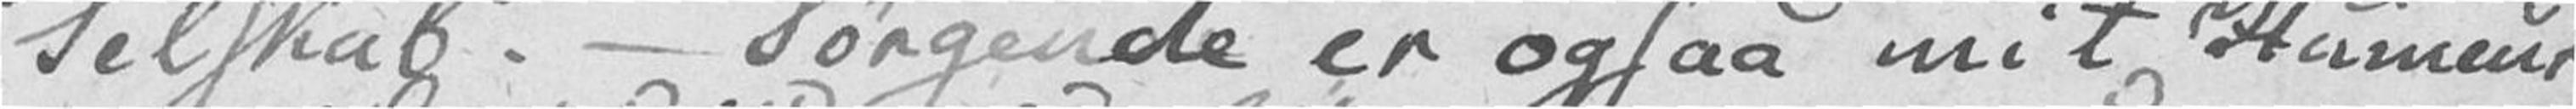Selskab. – Sørgende er ogsaa mit Humeur
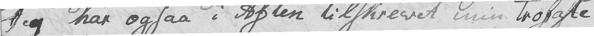Jeg har ogsaa i Aften tilskrevet min trofaste
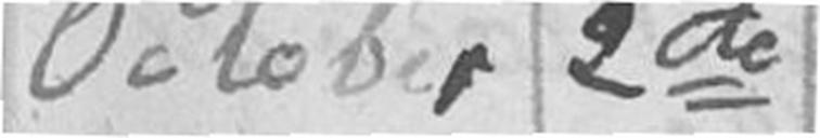October 2de
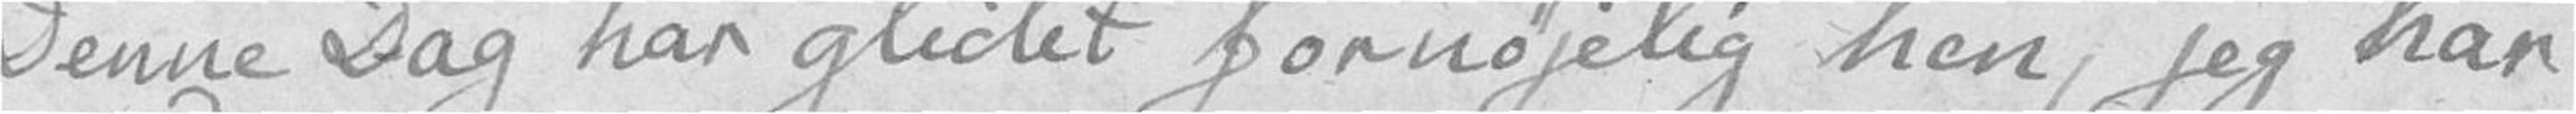Denne Dag har glidet fornøjelig hen, jeg har
